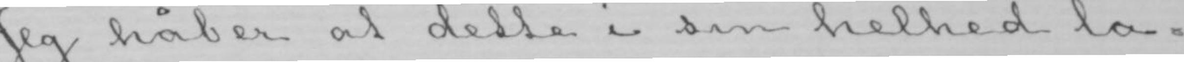Jeg håber at dette i sin helhed la-
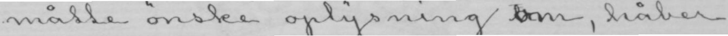måtte ønske oplysning hom, håber
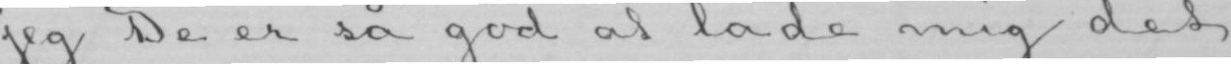jeg De er så god at lade mig det
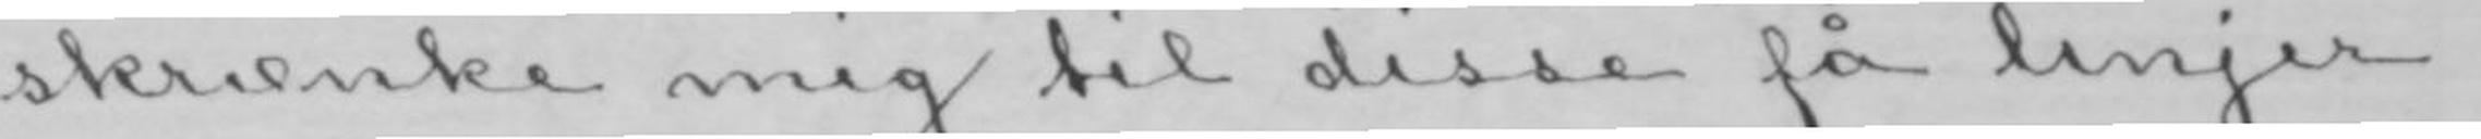skrænke mig til disse få linjer.
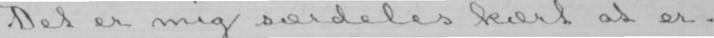Det er mig særdeles kært at er-
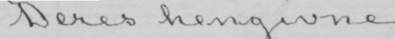Deres hengivne
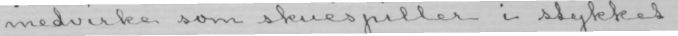medvirke som skuespiller i stykket.
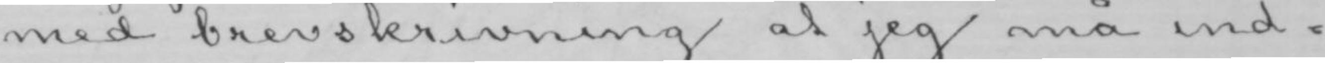med brevskrivning at jeg må ind-
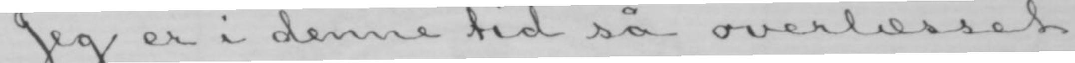Jeg er i denne tid så overlæsset
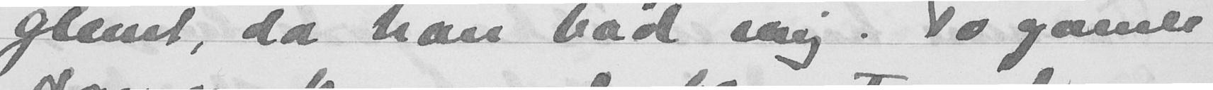glemt, da han bad mig. To gamle
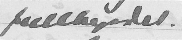fullbyrdet.
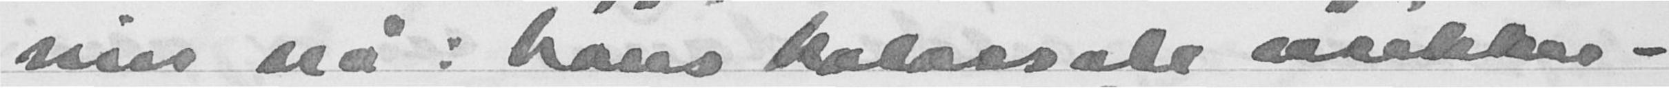inn nå; hans kolassale usikker-
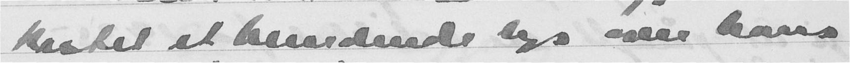kastet et blendende lys over hans
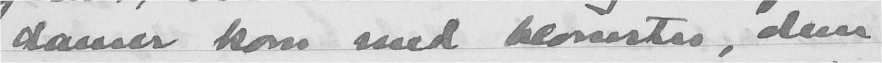damer kom med blomster, dem
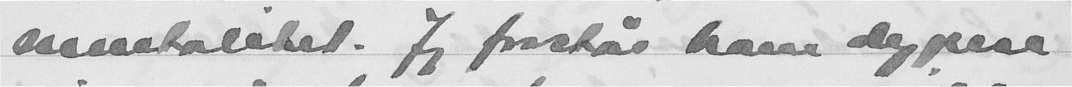mentalitet. Jeg forstår ham dypere
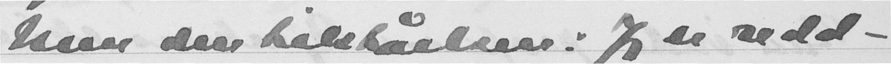Men den tilståelsen: Jeg er redd -
